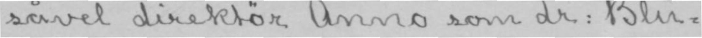såvel direktør Anno som dr: Blu-
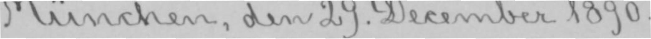München, den 29. December 1890.
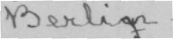Berlin.
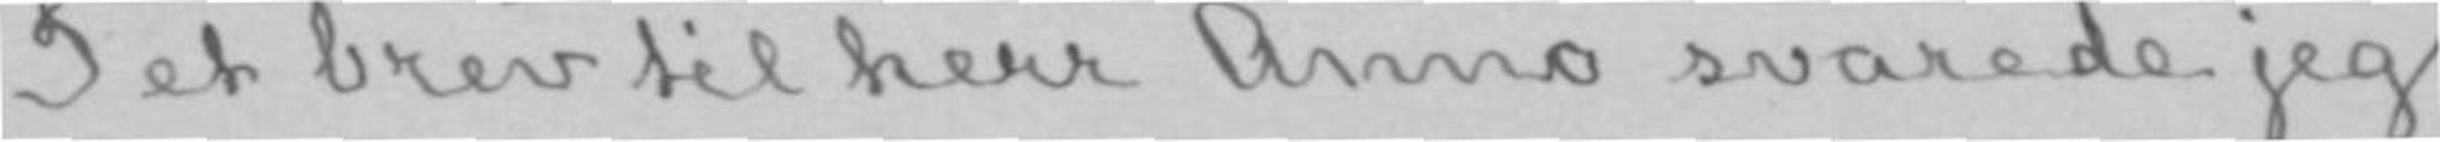I et brev til herr Anno svarede jeg
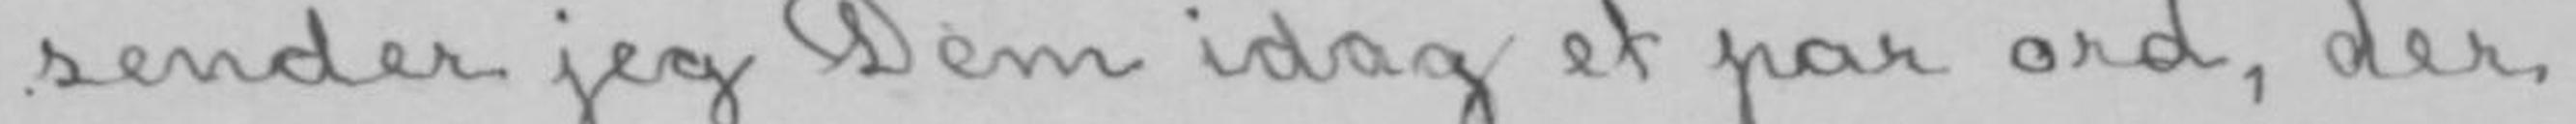sender jeg Dem idag et par ord, der
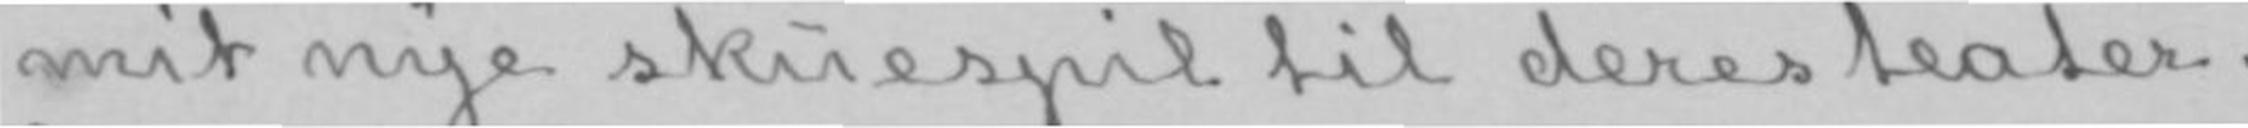mit nye skuespil til deres teater.
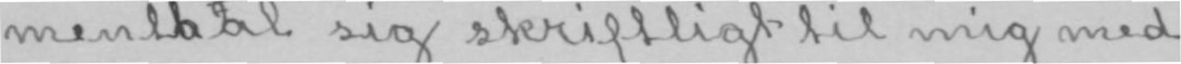mentahal sig skriftligt til mig med
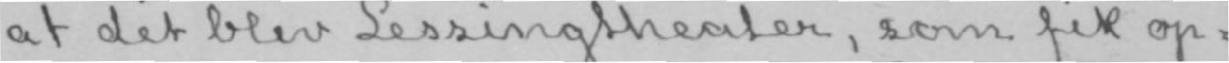at det blev Lessingtheater, som fik op-
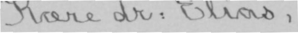Kære dr: Elias,
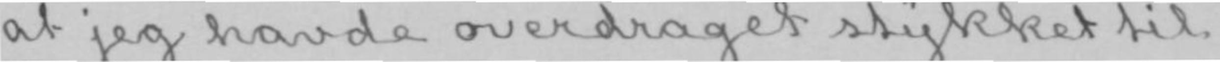at jeg havde overdraget stykket til
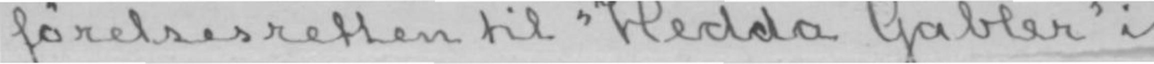førelsesretten til «Hedda Gabler» i
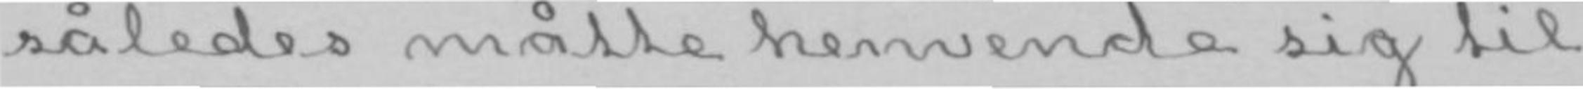således måtte henvende sig til
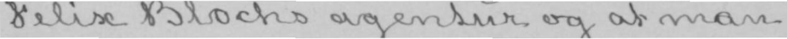Felix Blochs agentur og at man
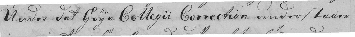Under det Høye Collegii Correction understaaer
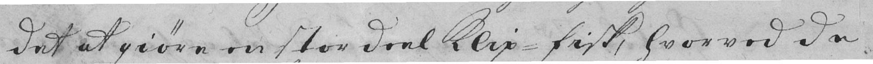det at giøre en stor deel Klip-fisk, hvorved de
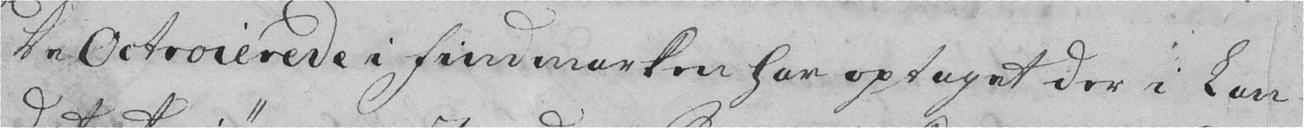De Octroierede i Findmarken har optaget der i Lan-
